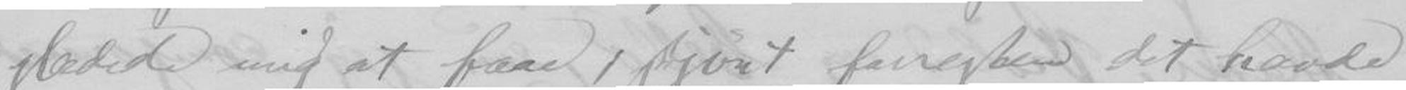glædede mig at faae, skjønt forresten det havde
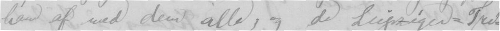ham af med dem alle, og de Leipziger=Tri-
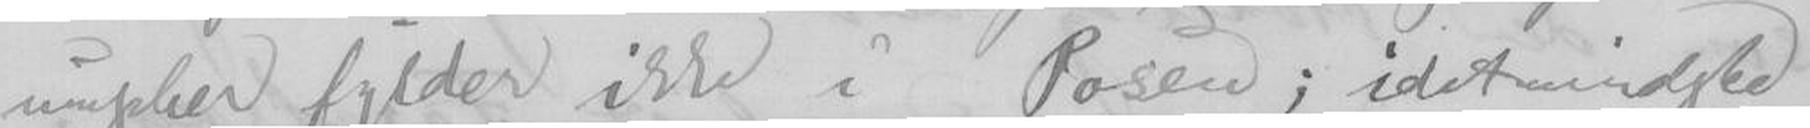umpher fylder ikke i Posen; idet mindste
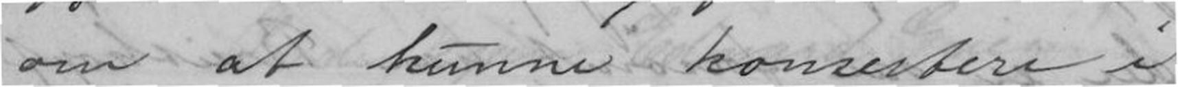om at kunne konsertere i
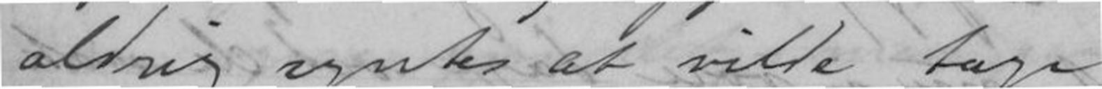aldrig syntes at vilde tage
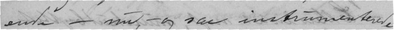ende, - nu, - og saa instrumenterede
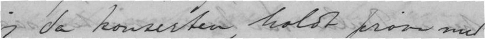da konserten, holdt prøve med
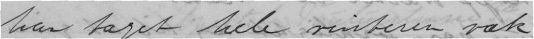har taget hele vinteren væk
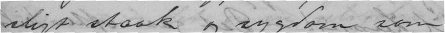sligt staak og sygdom som
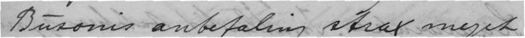Busonis anbefaling strax meget
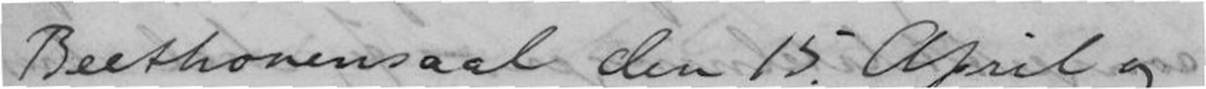Beethovensaal den 15 April og
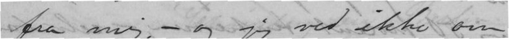fra mig, - og jeg ved ikke om
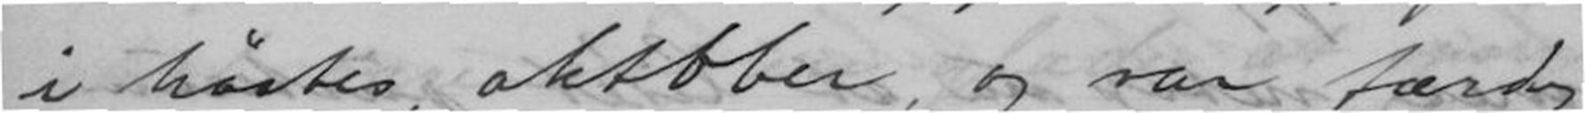i høstes, oktober, og var færdig
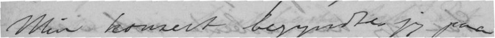Min konsert begyndte jeg paa
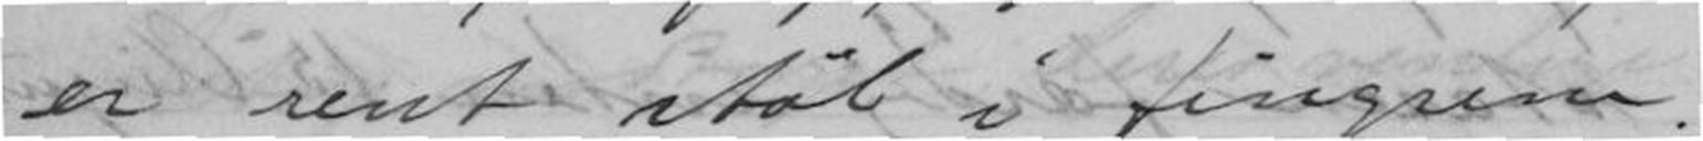er rent støl i fingrene.
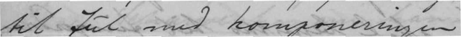til Jul med komponeringen,
